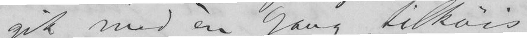gik med èn Gang tilkøis,
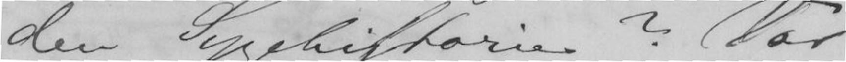den Sygehistorie? Var
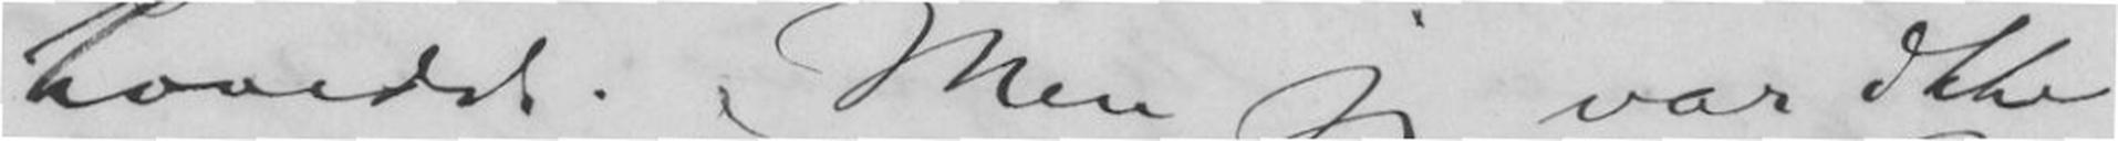hovedet. Men jeg var ikke
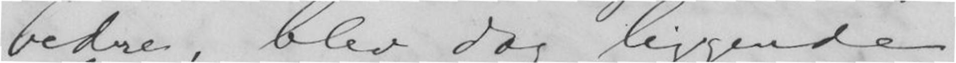bedre, blev dog liggende
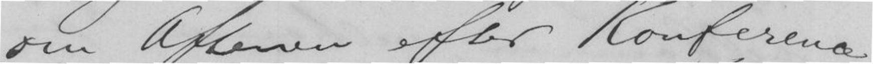om Aftenen efter Konferance
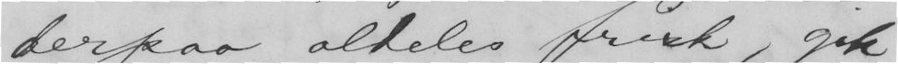derpaa aldeles frisk, gik
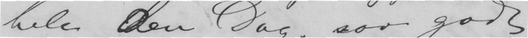hele den Dag, sov godt
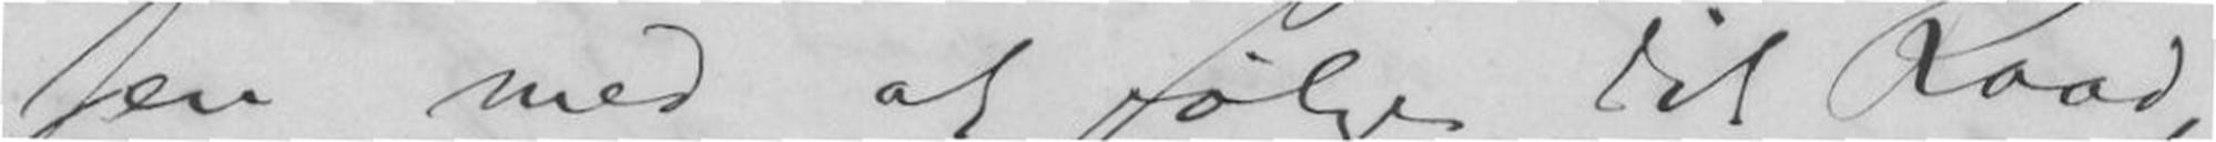sen med at følge dit Raad,
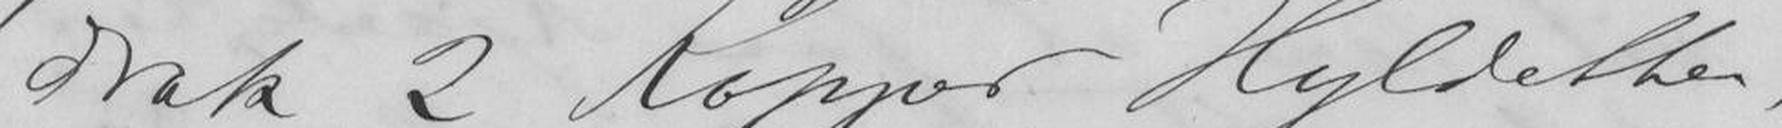drak 2 Kopper Hyldethe,
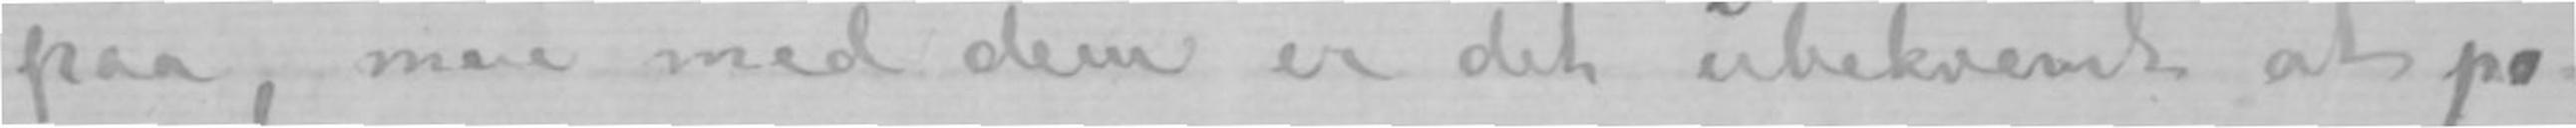paa, men med dem er det ubekvemt at po-
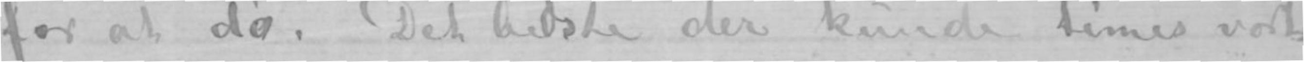for at dø. Det bedste der kunde times vort
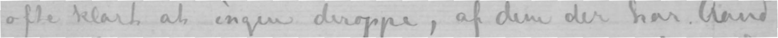ofte klart at ingen deroppe, af dem der har Aand
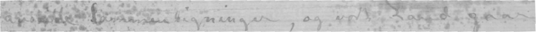anstille Sammenligninger, og vort Land gaar
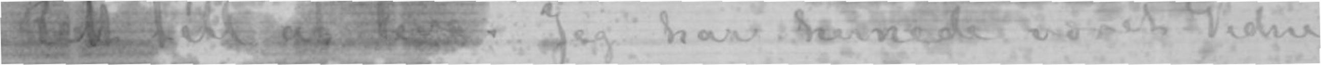Rett till at leve. Jeg har hernede været Vidne
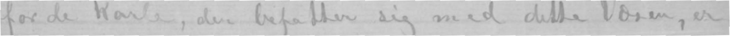for de Karle, der befatter sig med dette Væsen, er
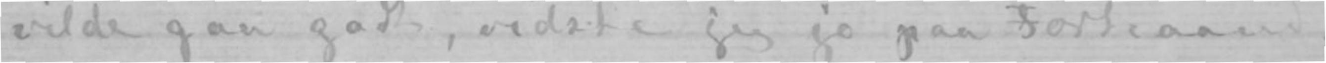vilde gaa godt, vidste jeg jo paa Forhaand.
